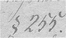§ 255.
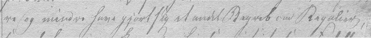re og mindre have gjort sig et andet Begreb om Regalier,
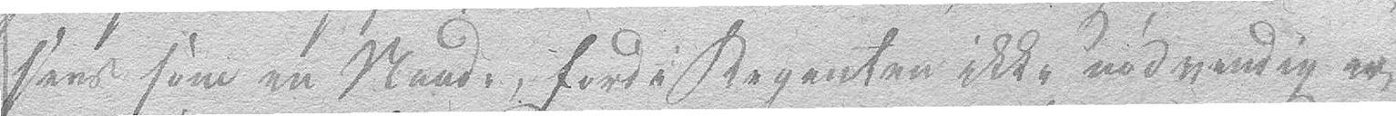sees som en Mand, fordi Regenten ikke nødvendig er
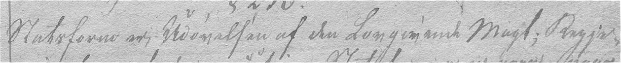Statsform er Udøvelsen af den Lovgivende Magt; Regje-
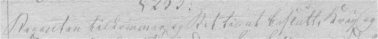Regenten tilkommer og Ret til at beslutte Krig og
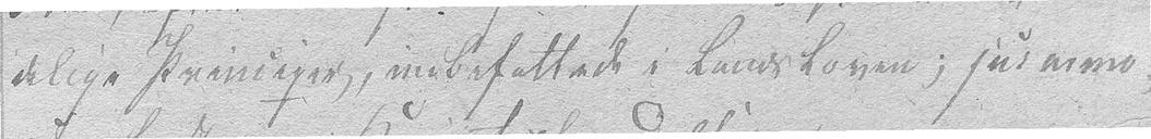delige Principer, indbefattede i Landsloven; jus armo-
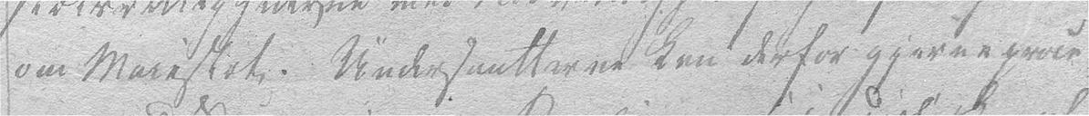om Maiestæt. Undersaatterne kan derfor gjerne proce-
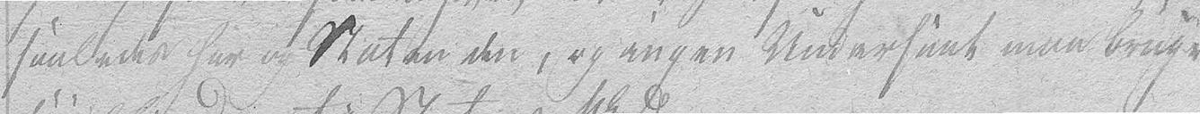saaledes har og Staten den, og ingen Undersaat maa bruge
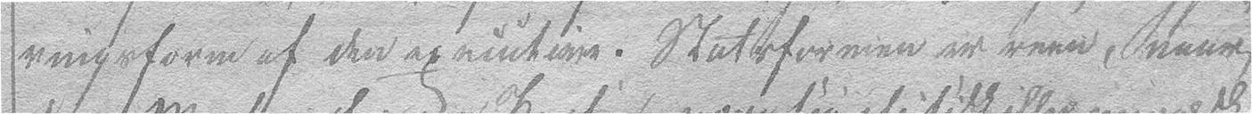ringsform af den executive. Statsformen er reen, naar
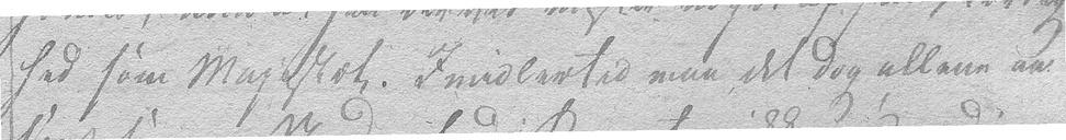hed som Majestæt. Imidlertid maa det dog allene an-
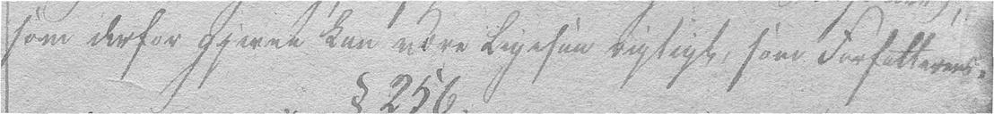som derfor gjerne kan være ligesaa rigtigt som Forfatterens.
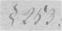§ 253.
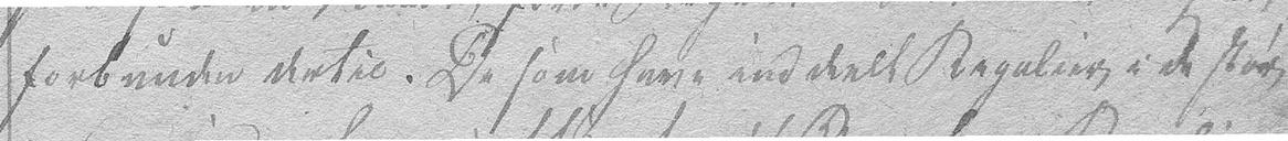forbunden dertil. De som have inddeelt Regalier i de stør-
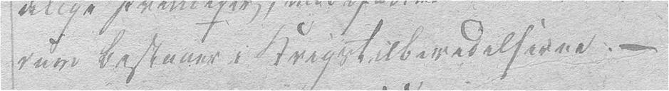rum bestaaer i Krigstilberedelserne. –
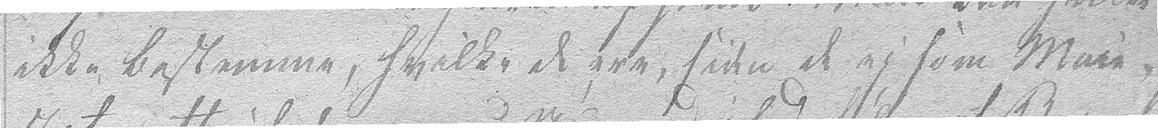ikke bestemme, hvilke de ere, siden de ey som Maie-
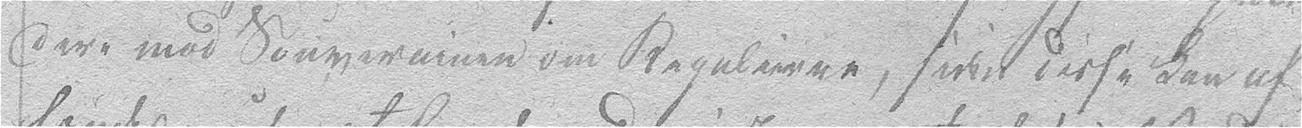dere mod Souverainen om Regalierne, siden disse kan af-
